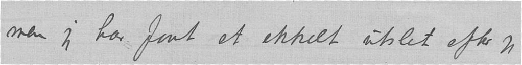men jeg har faat et ekkelt utslet efter jeg
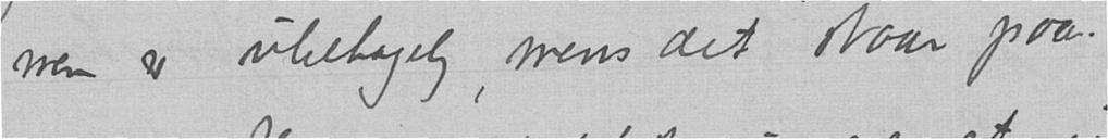men er ubehagelig, mens det staar paa.
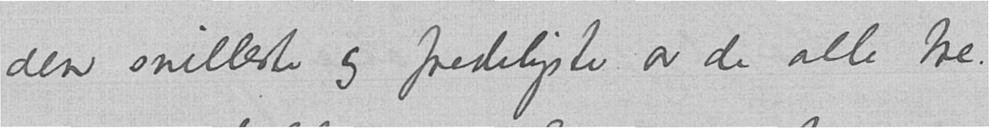den snilleste og fredeligste av de alle tre.
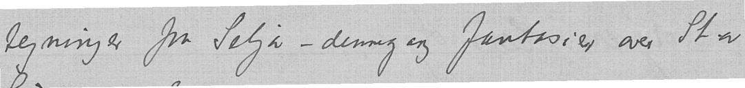tegninger fra Selja – dennegang fantasier over St.a
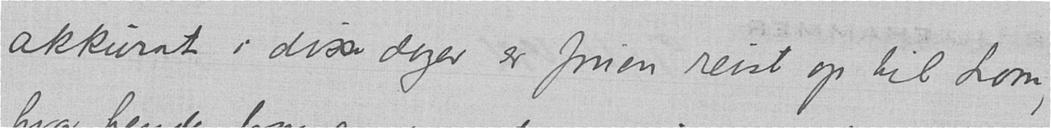akkurat i dise dager er fruen reist op til Lom,
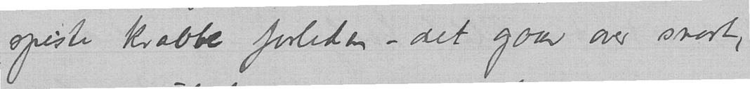spiste krabbe forleden – det gaar over snart,
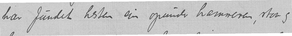har fundet hesten sin opunder hammeren, staar og
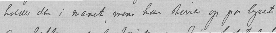holder den i manet, mens han stirrer op paa lyset
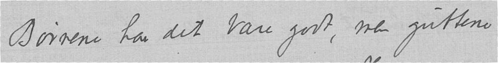Børnene har det bare godt, men guttene
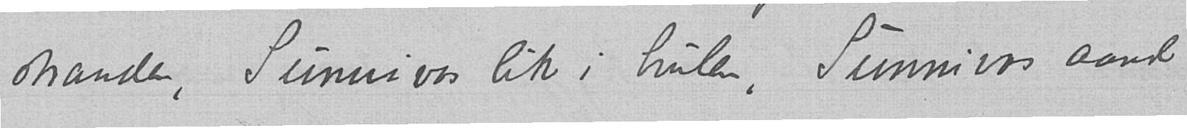stranden, Sunnivas lik i hulen, Sunnivas aand
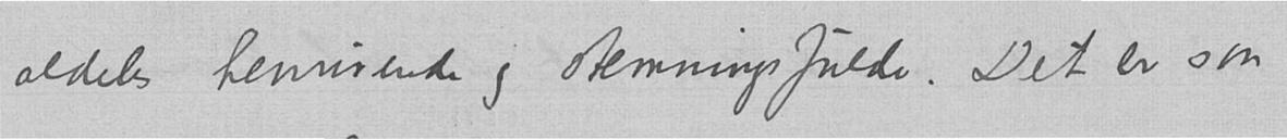aldeles henrivende og stemningsfulde. Det er saa
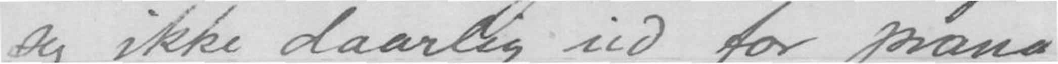seg ikke daarlig ud for piano
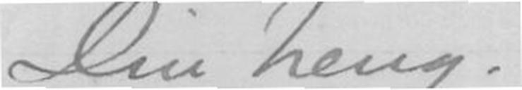Din heng.
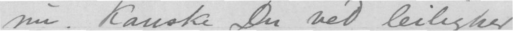nu. Kanske Du ved leilighed
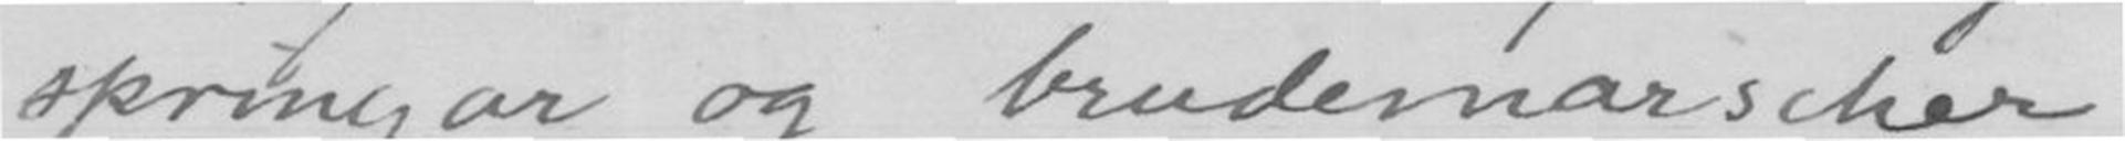springar og brudemarscher
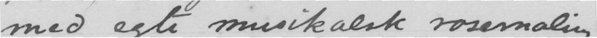med ægte musikalsk rosemaling
